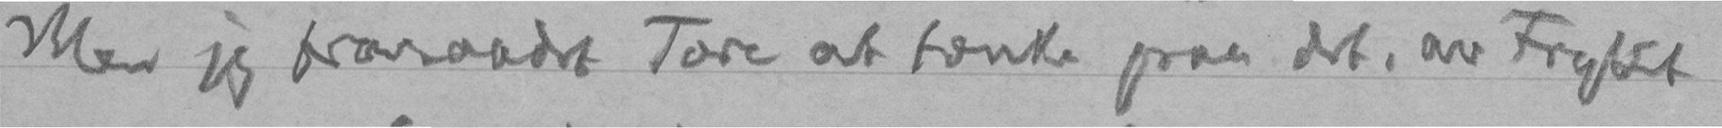Men jeg fraraadet Tore at tænke paa det, av Frykt
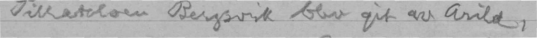Tillatelsen Bergsvik blev git av Arild,
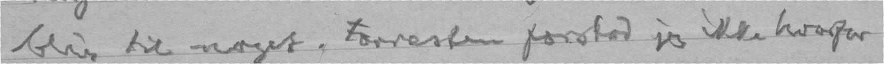blir til noget. Forresten forstod jeg ikke hvorfor
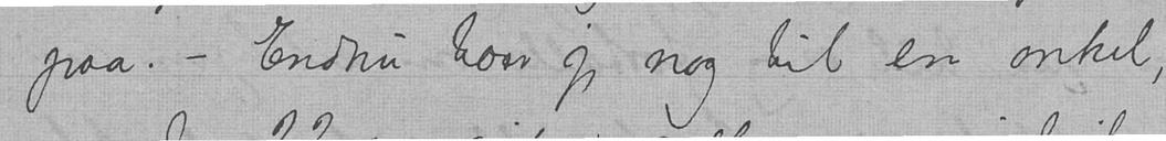paa. - Endnu bærer jeg nag til en onkel,
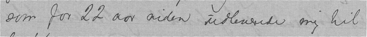som for 22 aar siden udleverede mig til
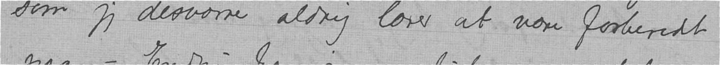som jeg desværre aldrig lærer at være forberedt
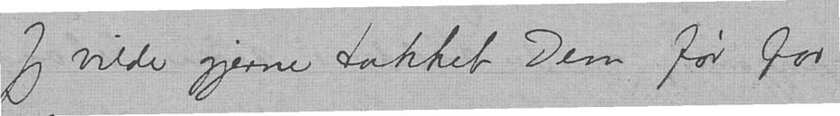Jeg vilde gjerne takket Dem før for
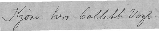Kjære herr Collett Vogt!
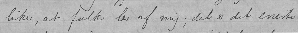like, at folk ler af mig; det er det eneste
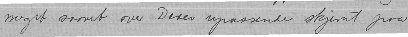meget saaret over Deres upassende skjemt paa
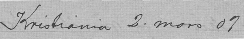Kristiania 2. mars 09
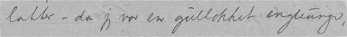latter - da jeg var en gullokket engleunge,
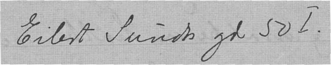Eilert Sundts gd 50 I.
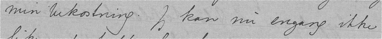min bekostning. Jeg kan nu engang ikke
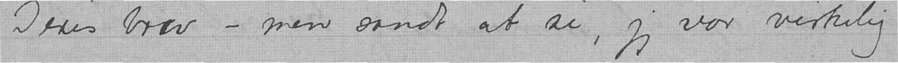Deres brev - men sandt at si, jeg var virkelig
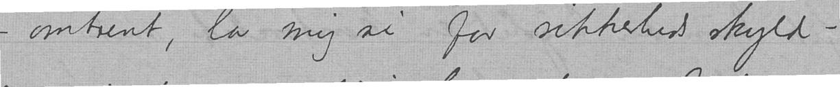- omtrent, la mig si for sikkerheds skyld -
